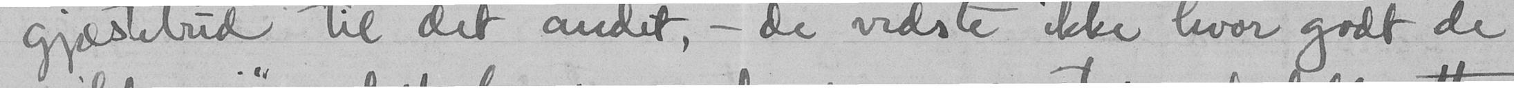gjæstebud til det andet, - de vidste ikke hvor godt de
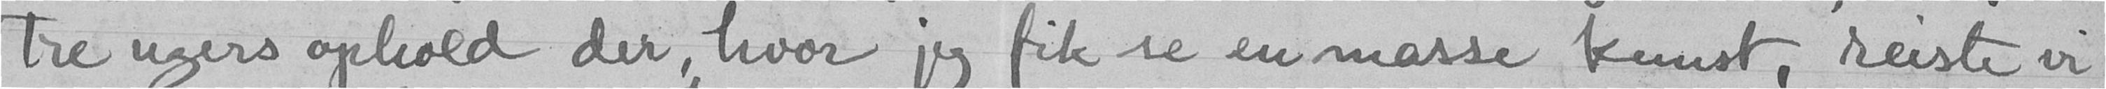tre ugers ophold der, hvor jeg fik se en masse kunst, reiste vi
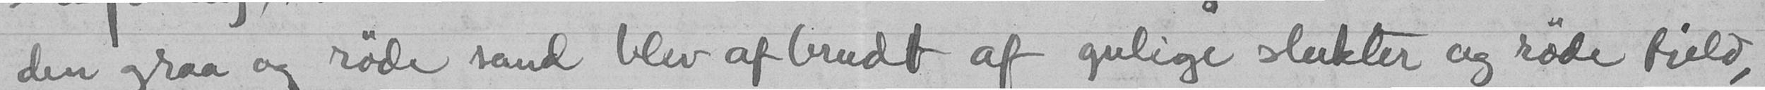den graa og røde sand blev af brudt af gulige slukter og røde fjeld,
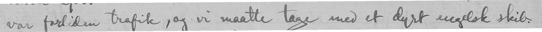var forliden trafik, og vi maatte tage med et dyrt engelsk skib.
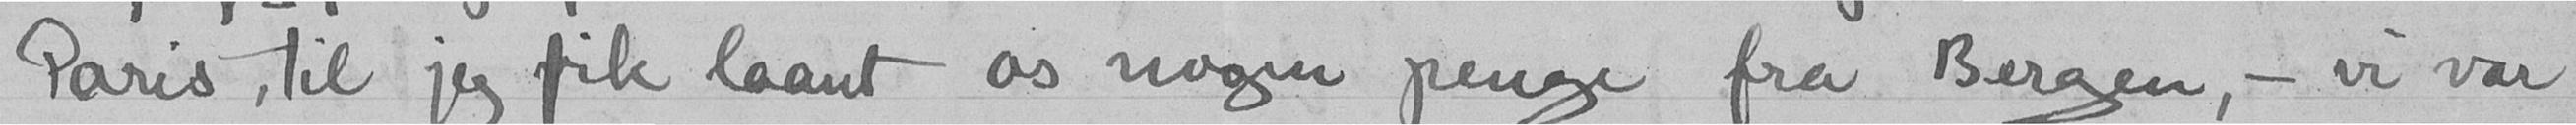Paris, til jeg fik laant os nogen penge fra Bergen, - vi var
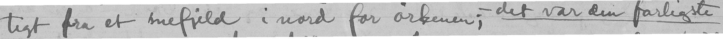tigt fra et snefjeld i nord for ørkenen, - det var den farligste
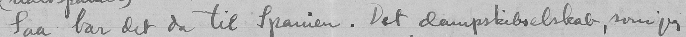Saa bar det da til Spanien. Det dampskibselskab, som jeg
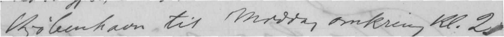Kjøbenhavn til Middag omkring Kl. 2.
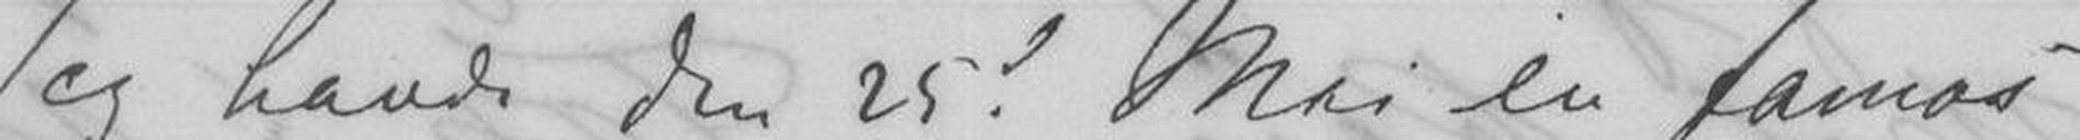Jeg havde den 25t Mai en famos
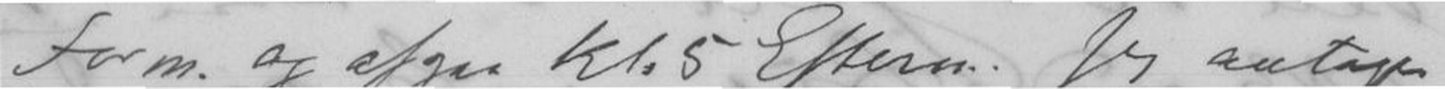Form. og afgaa Kl. 5 Efterm. Jeg antager
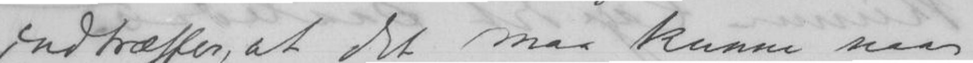indtræffer, at det maa kunne naa
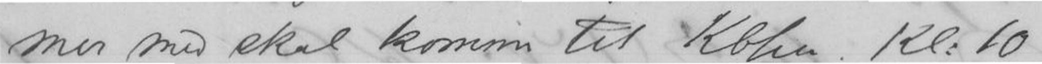mer med skal komme til Kbhn. Kl 10
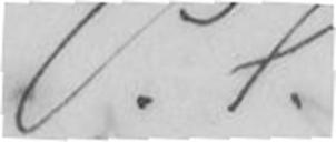P.S.
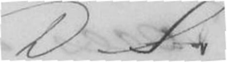D. S.
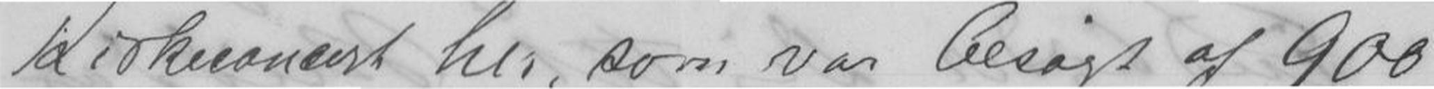Kirkeconcert her, som var besøgt af 900
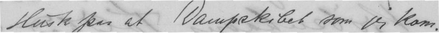Husk paa at Dampskibet som vi kom-
